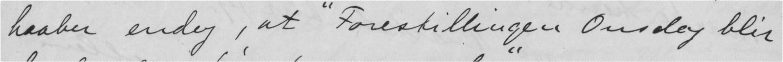haaber endog, at "Forestillingen Onsdag blir
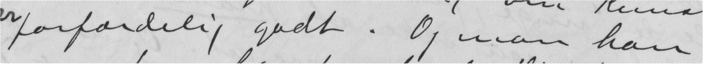forfærdelig godt. Og mon han
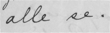alle se.
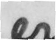er
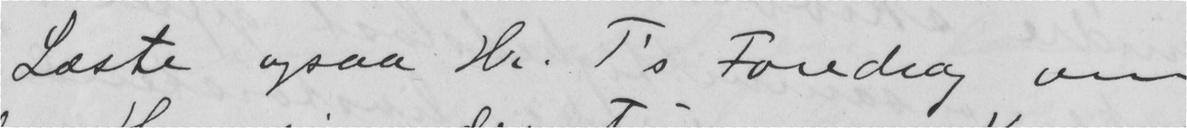Læste ogsaa Hr. T's Foredrag om
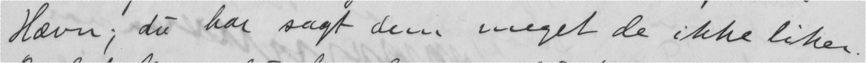Havn, du har sagt dem meget de ikke liker.
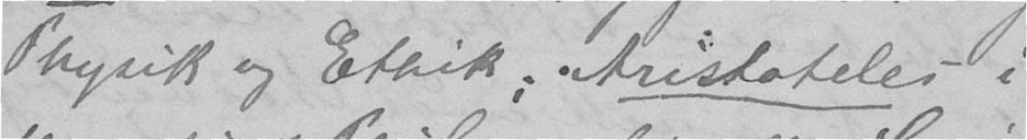Physik og Ethik, Aristoteles i
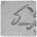54
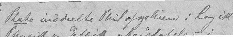Plato inddelte Philosophien i Logik,
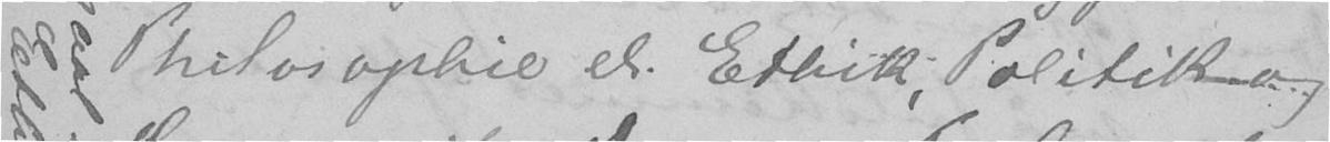Philosophie el. Ethik, Politik og
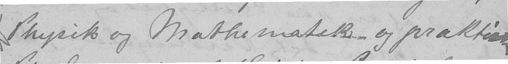Physik og Mathematik og praktisk
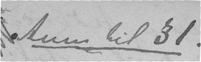Anm til § 1.
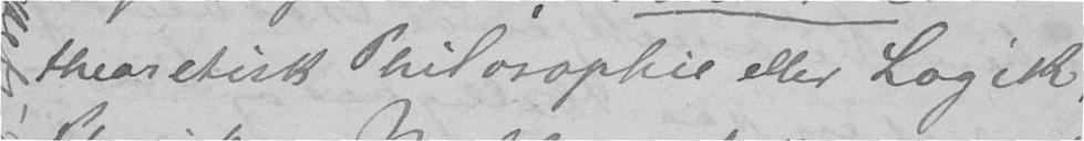theoretisk Philosophie eller Logik,
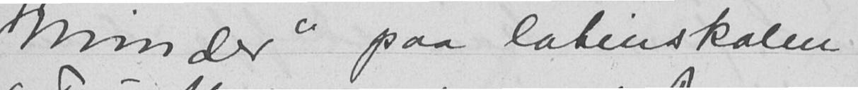Minder" paa latinskolen
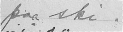paa ski.
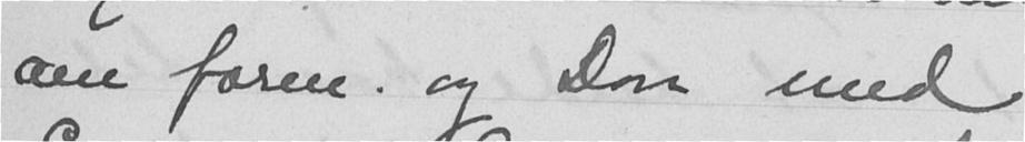om form. og Don med
